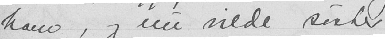ham, og nu vilde søster
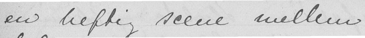en heftig scene mellem
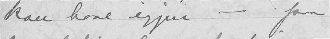kan have igjen - paa
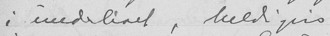i underlivet, heldigvis
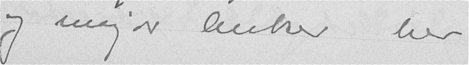og major Anker her
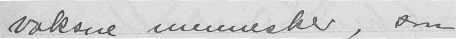voksne mennesker, som
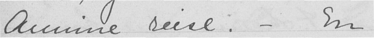Amine reise. - En
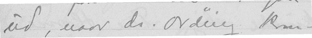ud, naar dr. Ording kom-
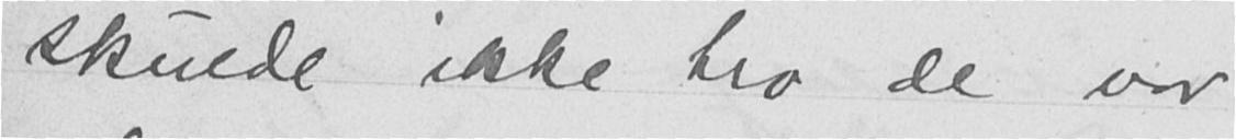skulde ikke tro de var
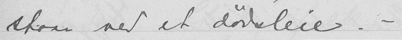staar ved et dødsleie. -
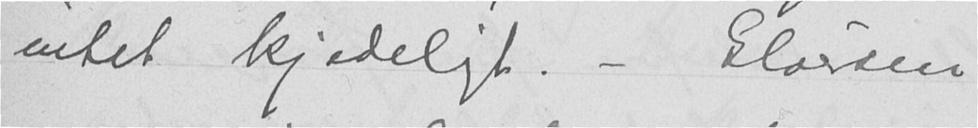intet kjedeligt. - Gløersen
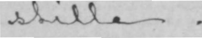stille.
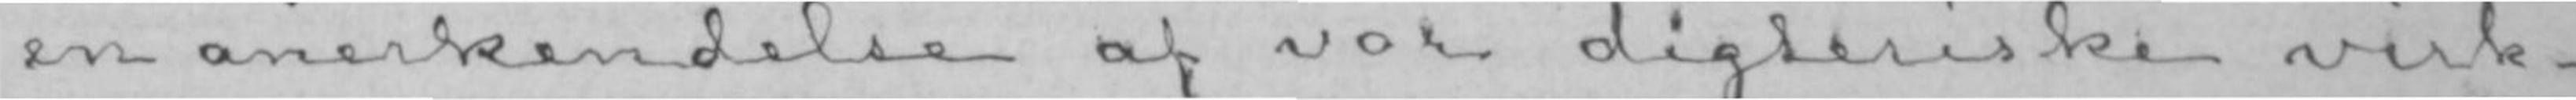offentlig anerkendelse af vor digteriske virk-
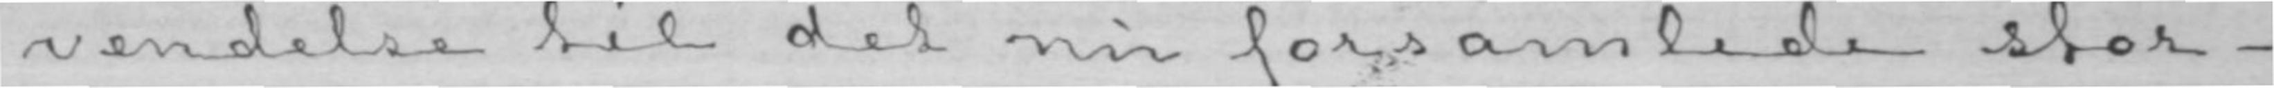vendelse til det nu forsamlede stor-
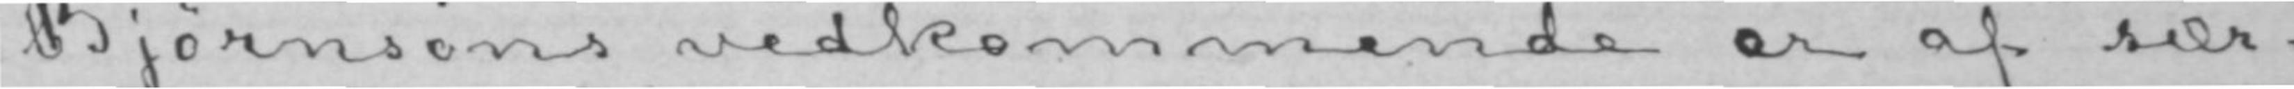Bjørnsons vedkommende er af sær
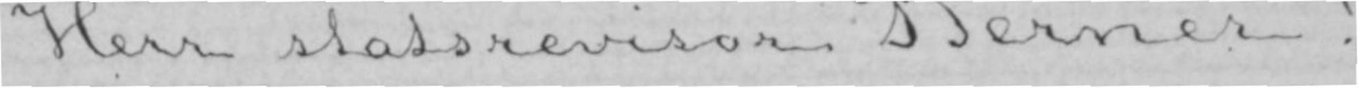Herr statsrevisor Berner!
